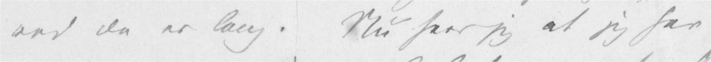ved Du er lang. Nu seer jeg at jeg har
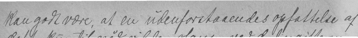kan godt være, at en udenforstaaendes opfattelse af
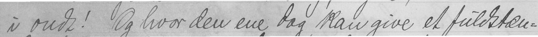i ondt! Og hvor den ene dag kan give et fuldstæn-
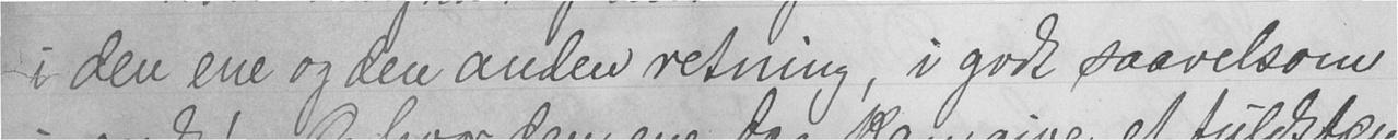i den ene og den anden retning, i godt saavelsom
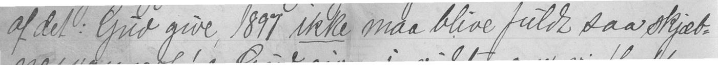af det: Gud give, 1879 ikke maa blive fuldt saa skjæb-
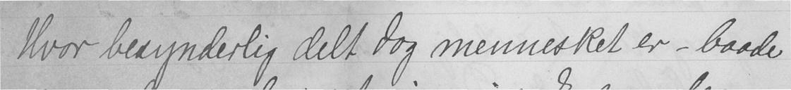Hvor besynderlig delt dog mennesket er - baade
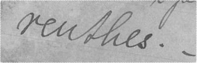renthes. -
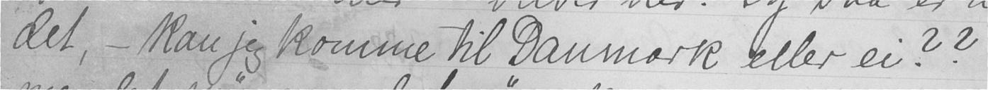det, - kan jeg komme til Danmark eller ei??
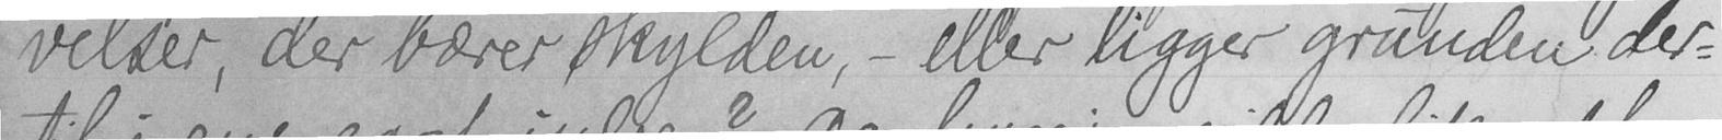velser, der bærer skylden, - eller ligger grunden der-
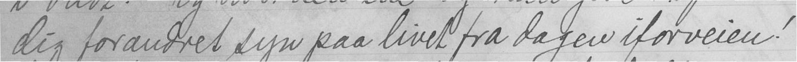dig forandret syn paa livet fra dagen iforveien!
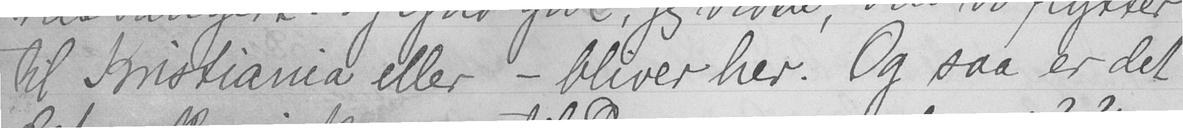til Kristiania eller - bliver her. Og saa er det
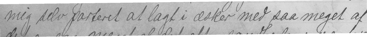mig selv parteret at lagt i æsker med saa meget af
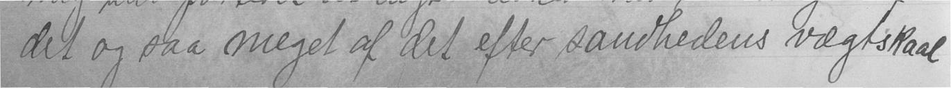det og saa meget af det efter sandhedens vægtskaal
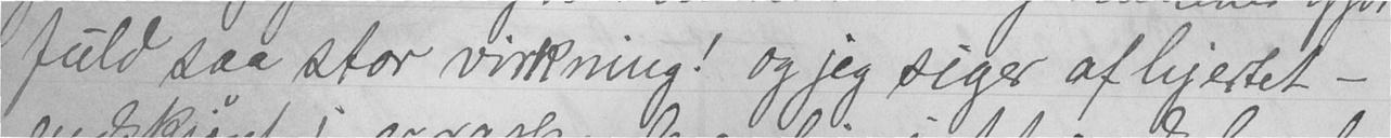fuld saa stor virkning! og jeg siger af hjertet -
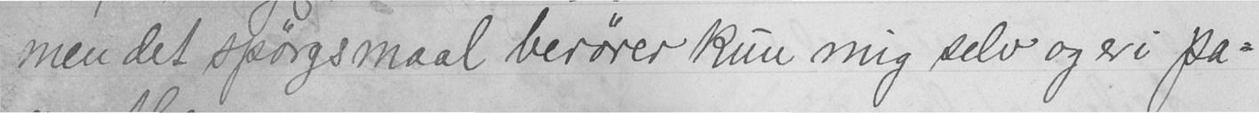men det spørgsmaal berører kun mig selv og er i pa-
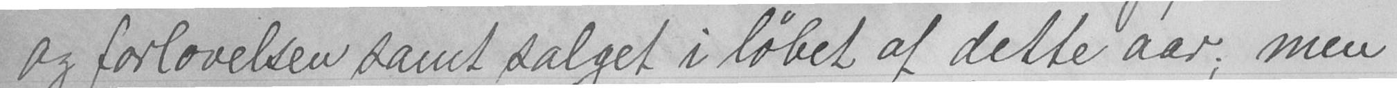og forlovelsen samt salget i løbet af dette aar; men
